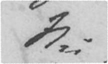Nu
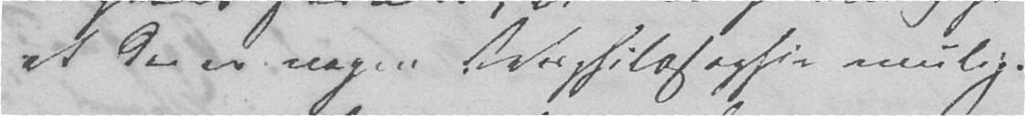at der er nogen Retsphilosophie mulig.
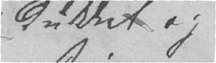dukke op
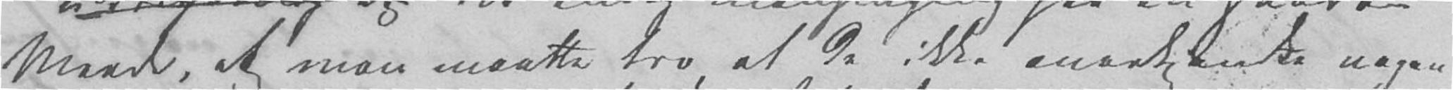Maade, at man maatte tro at de ikke anerkjendte nogen
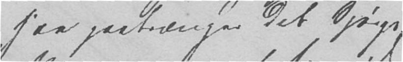saa paatrænger det Spørgs-
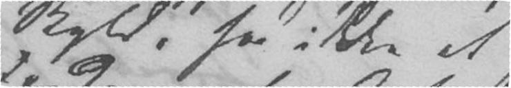Skyld, for ikke at
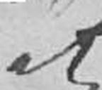at
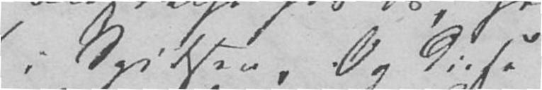i Spidsen. Og disse
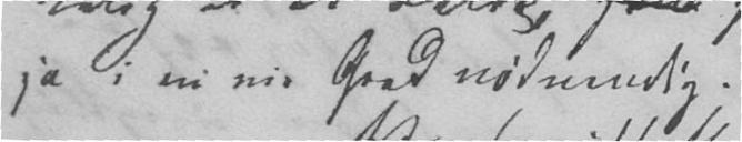ja i en vis Grad nødvendig.
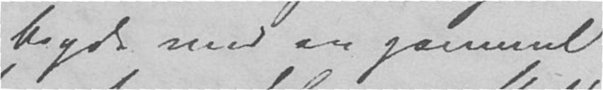bryde med en gammel
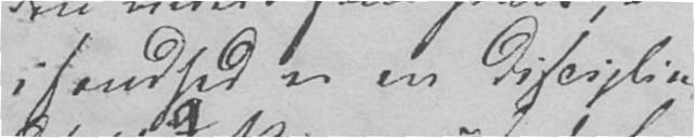i Sandhed er en Disciplin
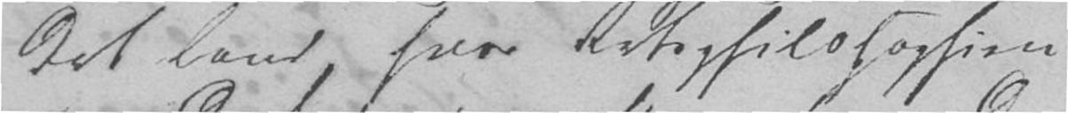det Land hvor Retsphilosophien
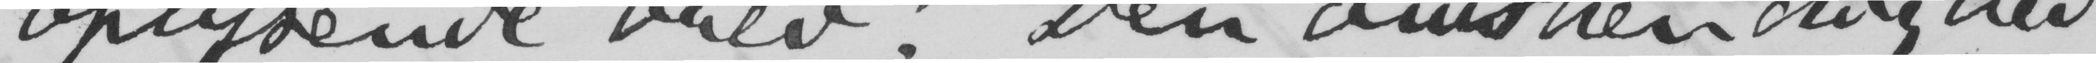oplysende brev! Den omstendighed,
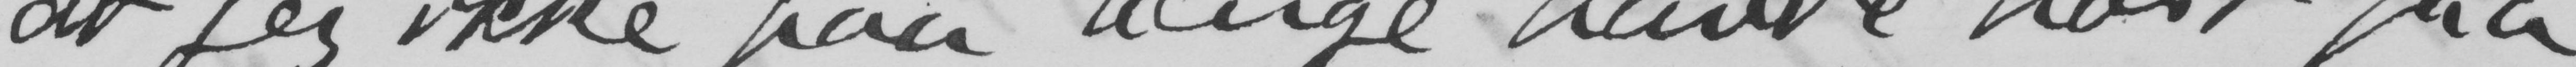at jeg ikke paa længe havde hørt fra
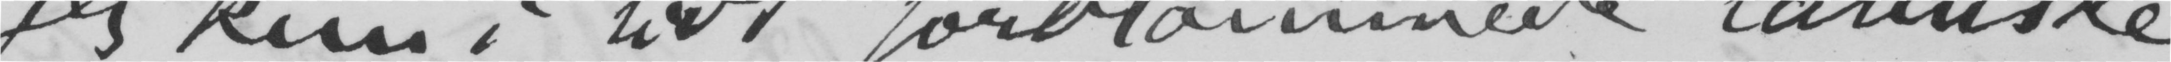jeg kun i lidt forblommende latinske
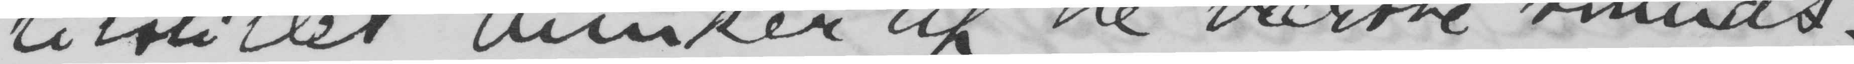tilstillet bunker af de værste smuds-
